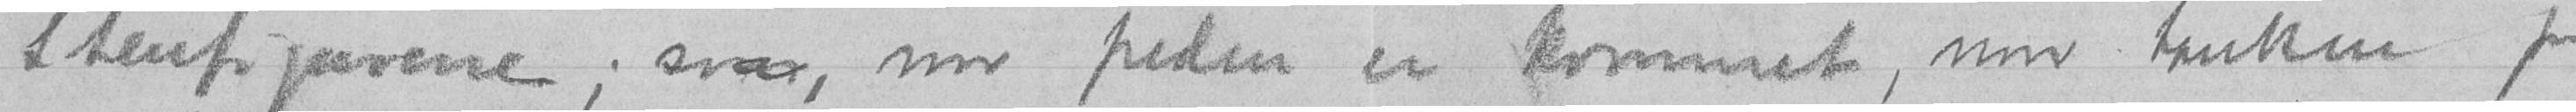stenfigurene; svar, naar freden er kommet, naar tanken paa
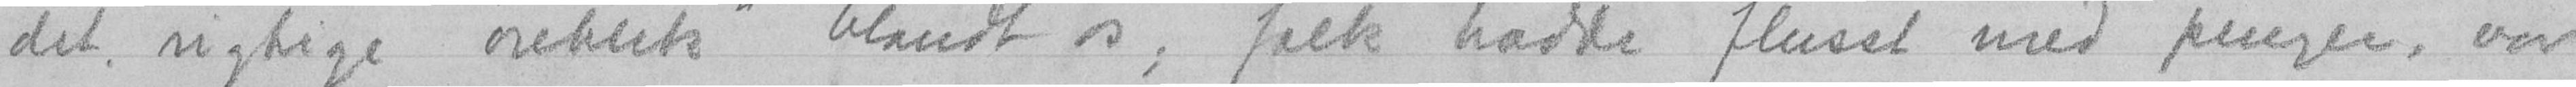det rigtige „øieblik” blandt os; folk hadde flusst med penger, var
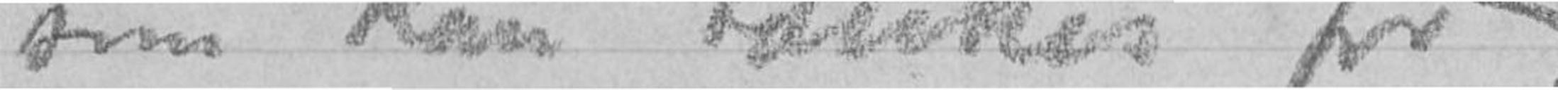som kan tankes for
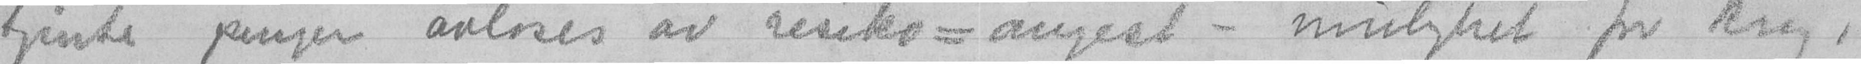tjente penger avløses av resiko-angst - mulighet for krig,
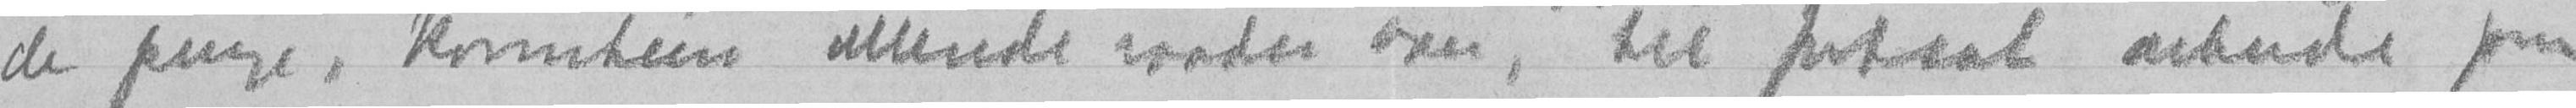de penge, komitéen allerede raader over, til fortsat arbeide paa
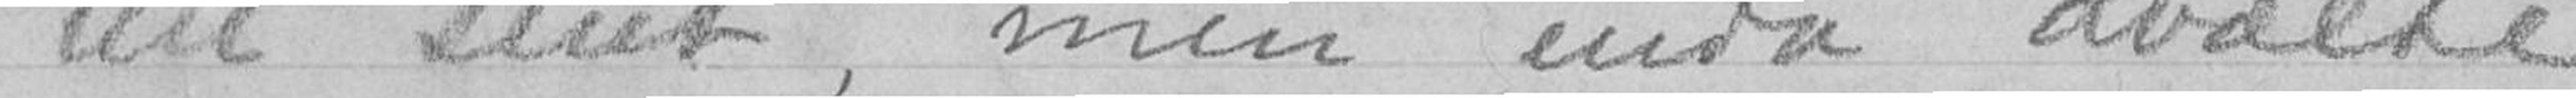litt sent, men enda dvælte
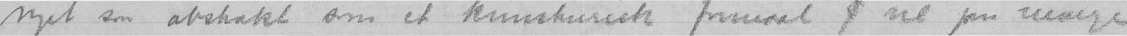noget saa abstrakt som et kunstnerisk formaal vil paa mange
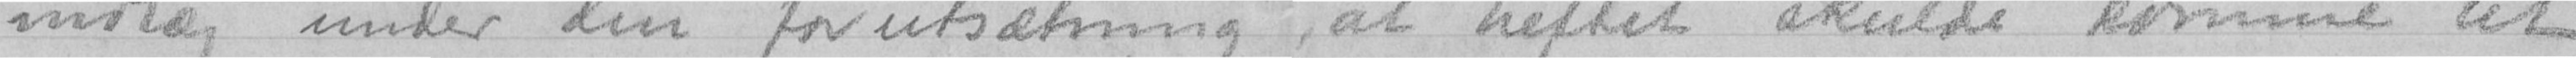indlæg under den forutsetning at heftet skulde komme ut
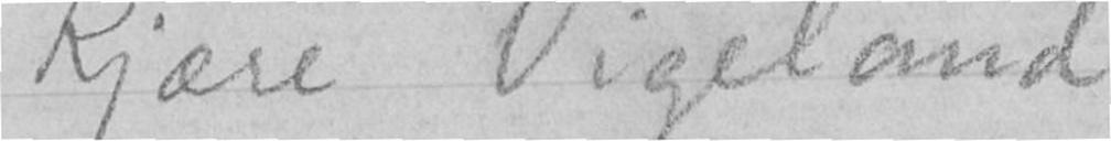Kjære Vigeland
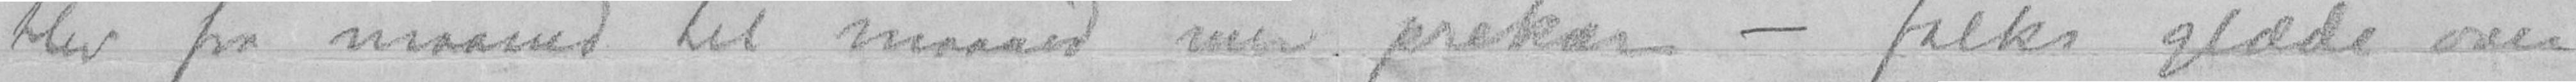blev fra maaned til maaned mer prekær - folks glæde over
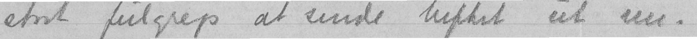stort feilgrep at sende heftet ut nu.
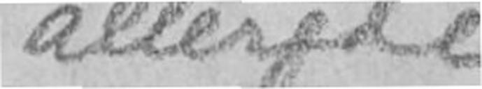allerede
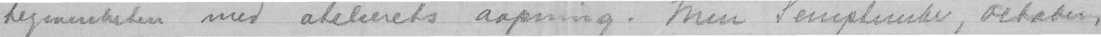begivenheten med atelierets aapning. Men September, October,
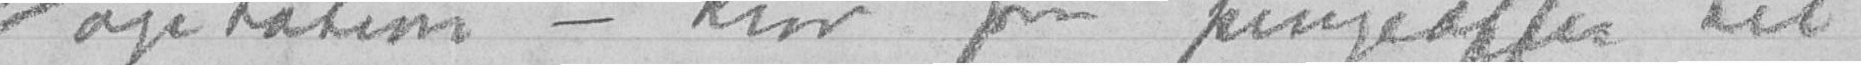agitation - krav paa pengeoffer til
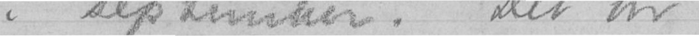i september. Det var
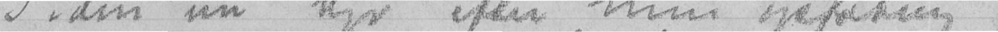Tiden nu byr efter min opfatning
